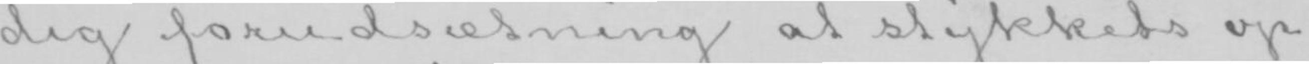dig forudsætning at stykkets op-
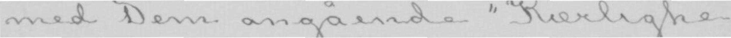med Dem angående «Kærlighe-
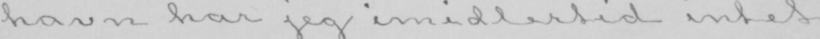havn har jeg imidlertid intet
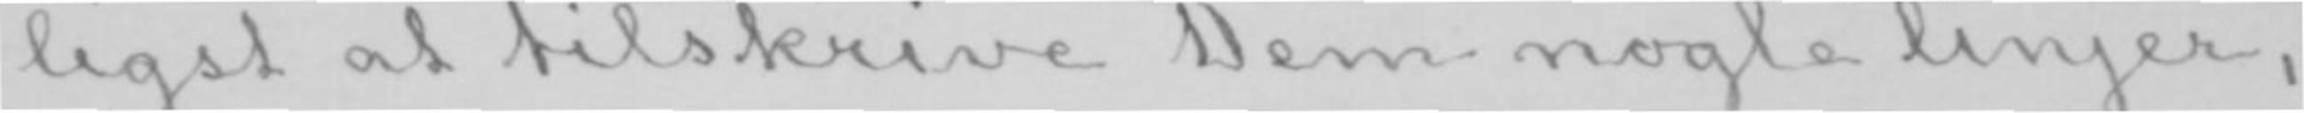ligst at tilskrive Dem nogle linjer,
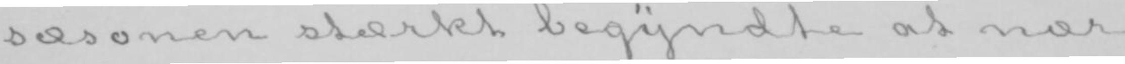sæsonen stærkt begyndte at nær-
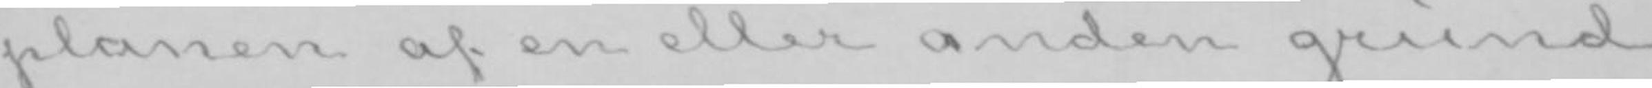planen af en eller anden grund
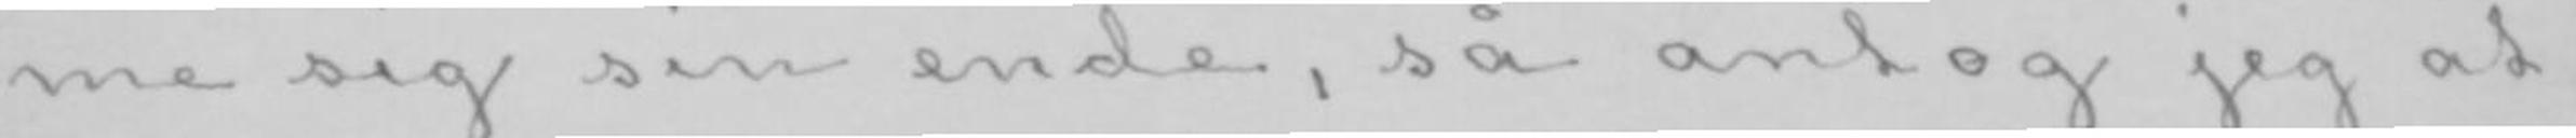me sig sin ende, så antog jeg at
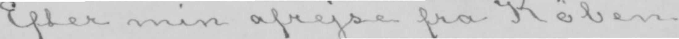Efter min afrejse fra Køben-
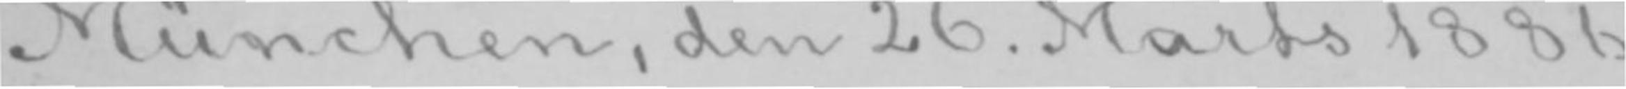München, den 26. Marts 1886.
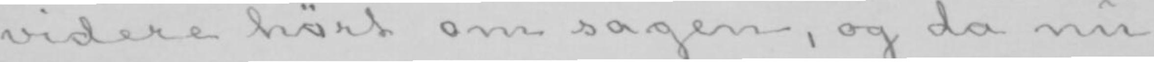videre hørt om sagen, og da nu
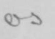os.
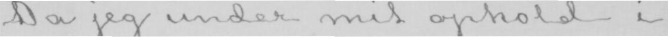Da jeg under mit ophold i
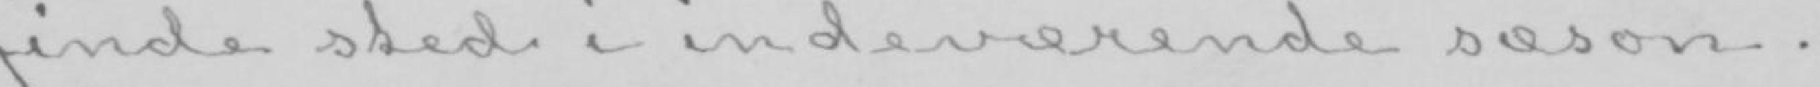finde sted i indeværende sæson.
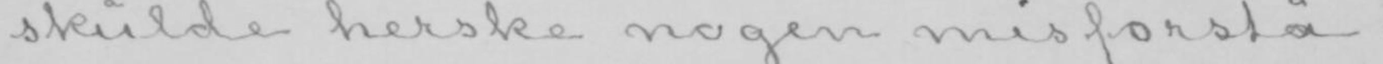skulde herske nogen misforstå-
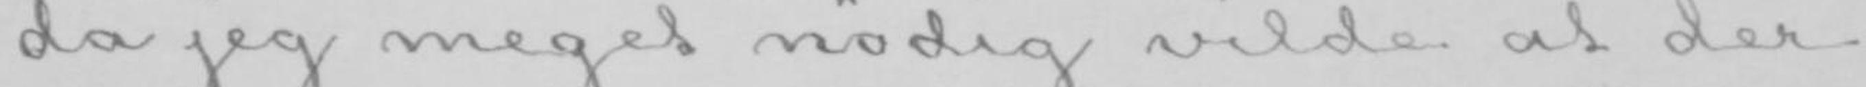da jeg meget nødig vilde at der
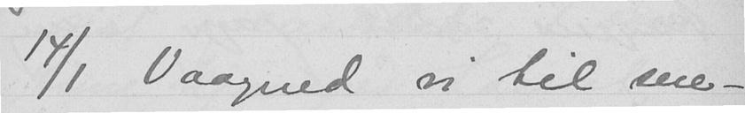14/1 Vaagned vi til sne -
